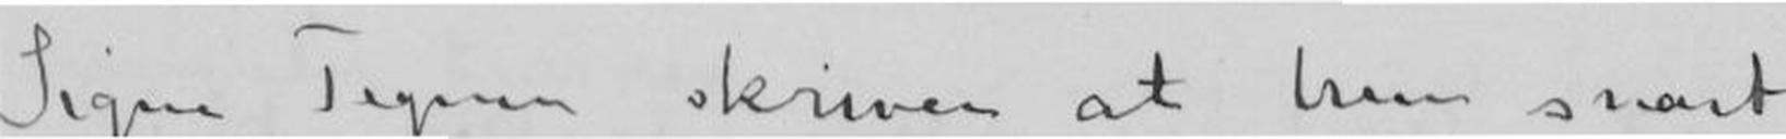Signe Tegen skriver, at hun snart
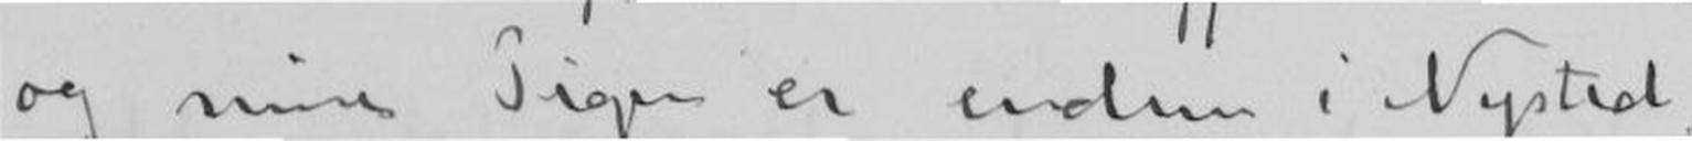og mine piger er endun i Nysted
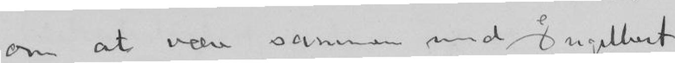om at være sammen med Engelhart
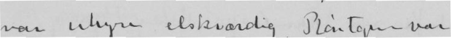var uhyre elskværdig, Röntgen var
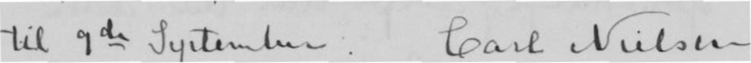til 9de September. Carl Nielsen
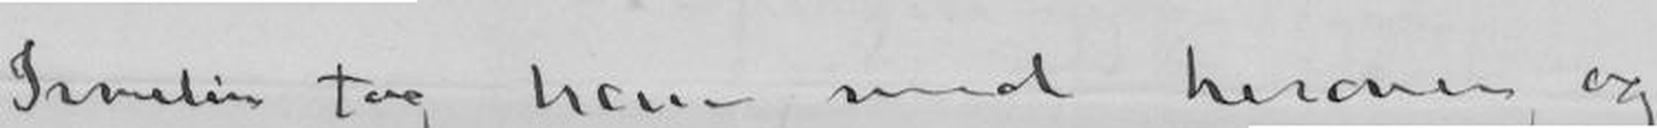Irmelin tog han med herover, og
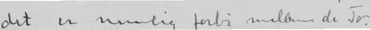det er mulig forbi mellom de To.
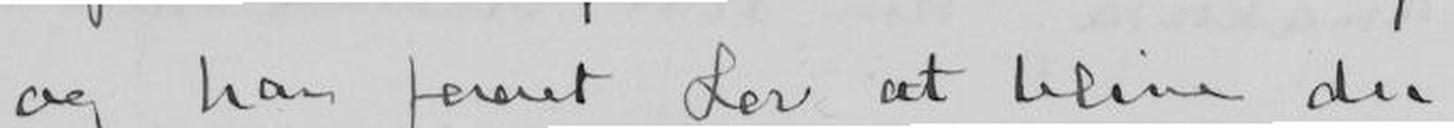og har faaet Dem at blive der
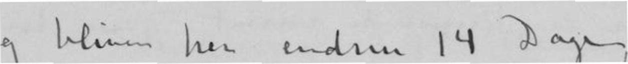Jeg blive her endun 14 Dage,
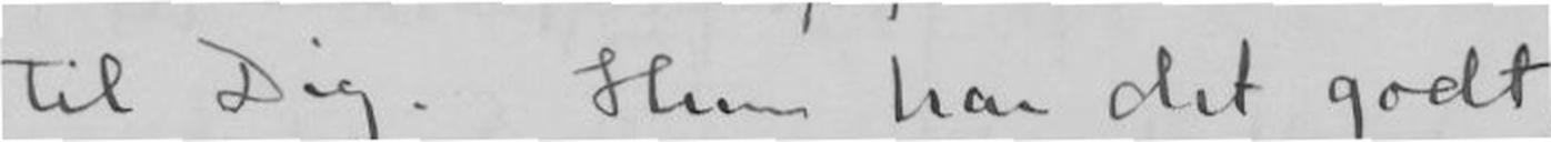til Dig Hun har det godt,
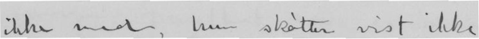ikke med, hun skøtter vist ikke
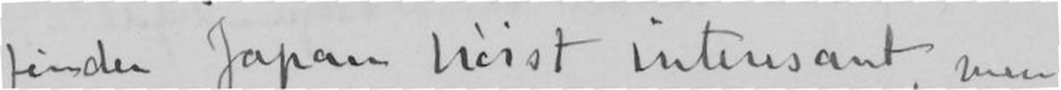finder Japan høist interesant, men
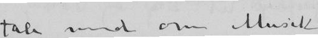tale med om Musik.
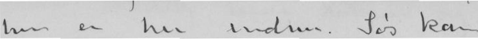han er her endun, So`s kom
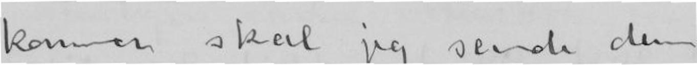kommer skal jeg sende dem
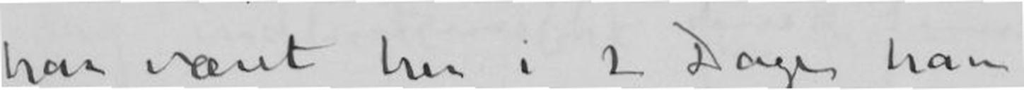har været her i 2 Dage han
